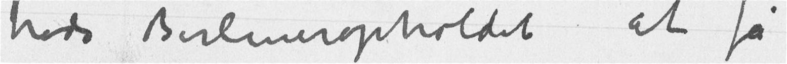trods Berlineropholdet at få
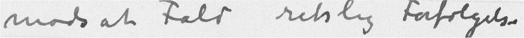modsat Fald retslig Forfølgelse
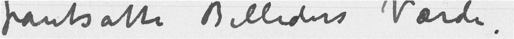pantsatte Billeders Værdi.
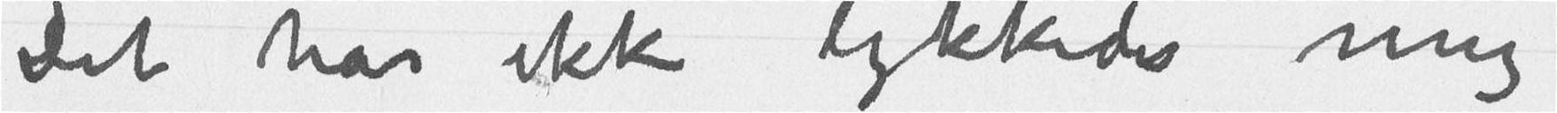Det har ikke lykkedes mig
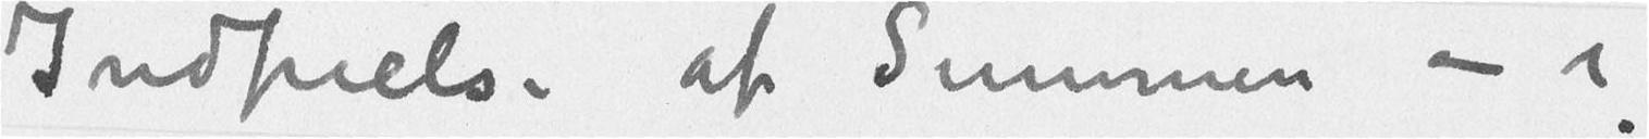Indfrielse af Summen – i
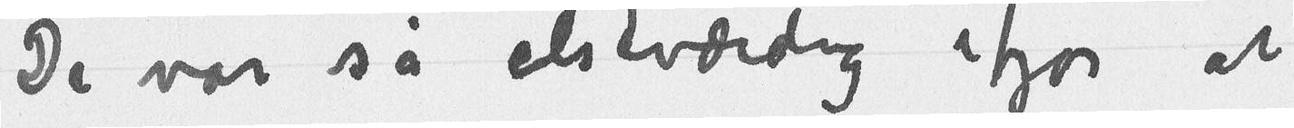De var så elskværdig ifjor at
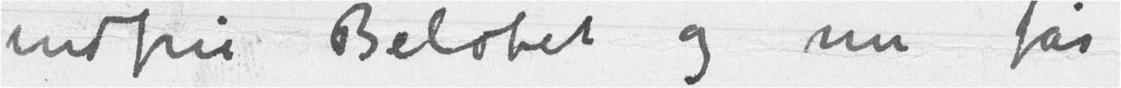indfrie Beløbet og nu får
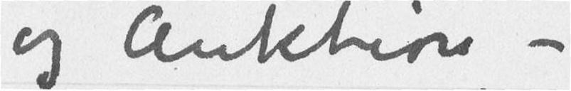og Auktion –
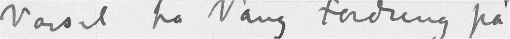Varsel fra Vang Fordring på
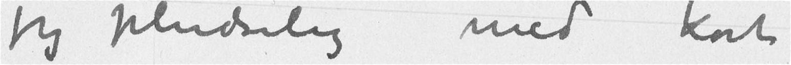jeg pludselig med kort
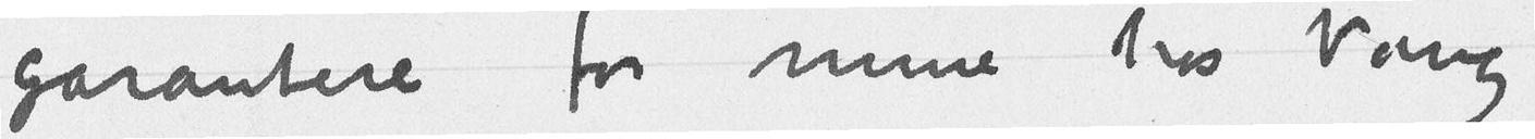garantere for mine hos Vang
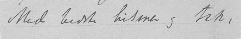Med bedste hilsener og tak,
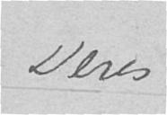Deres
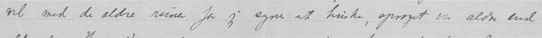vel med de ældre runer for jeg synes at huske, sproget var ældre end
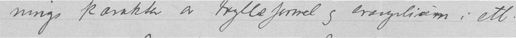nings karakter av trylleformel og evangelium i ett.
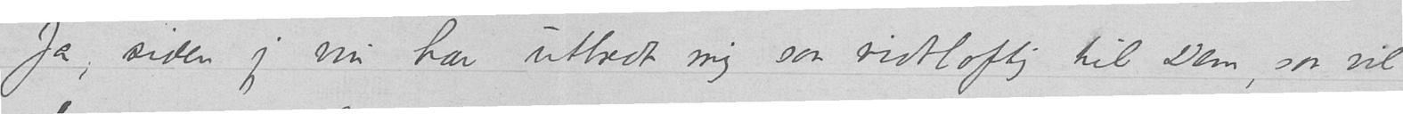– Ja, siden jeg nu har utbredt mig saa vidtløftig til Dem, saa vil
